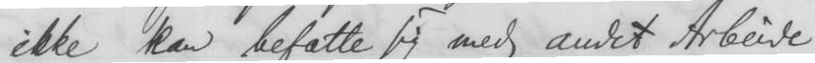ikke kan befatte sig med andet Arbeide.
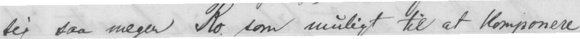sig saa megen Ro som muligt til at Komponere,
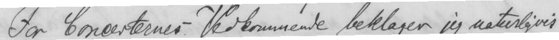For Concerternes Vedkommende beklager jeg naturligvis
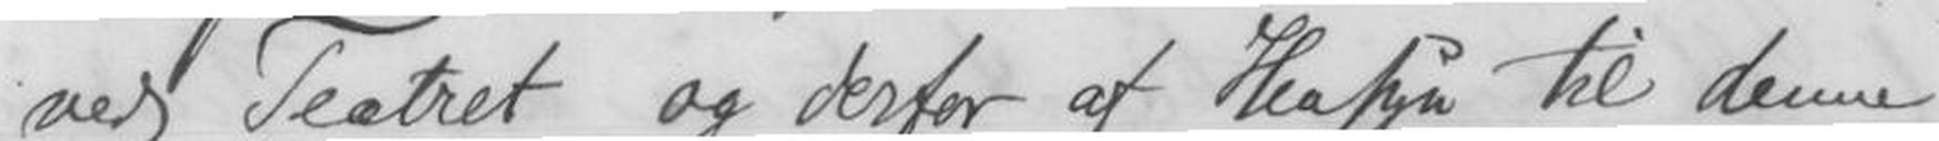ved Teatret og derfor af til denne
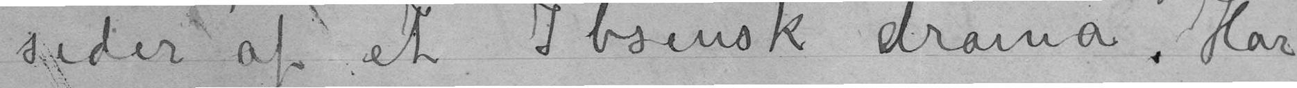sider af et Ibsensk drama. Har
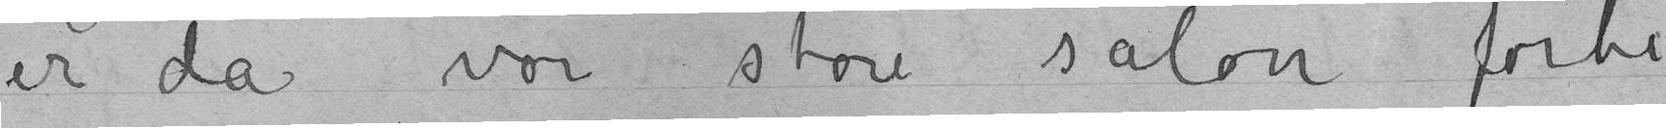er da vor store salon forbi
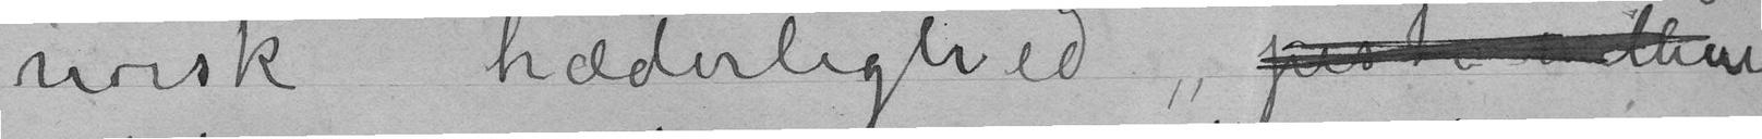norsk hæderlighed
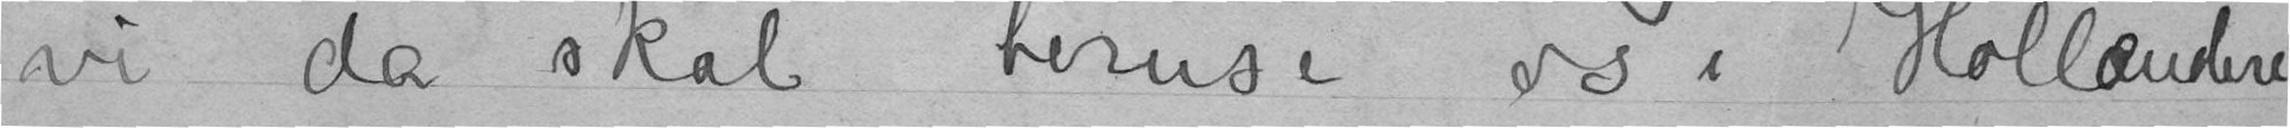vi da skal beruse os i Hollændere
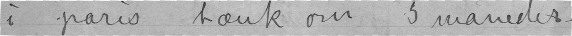i paris tænk om 5 måneder!
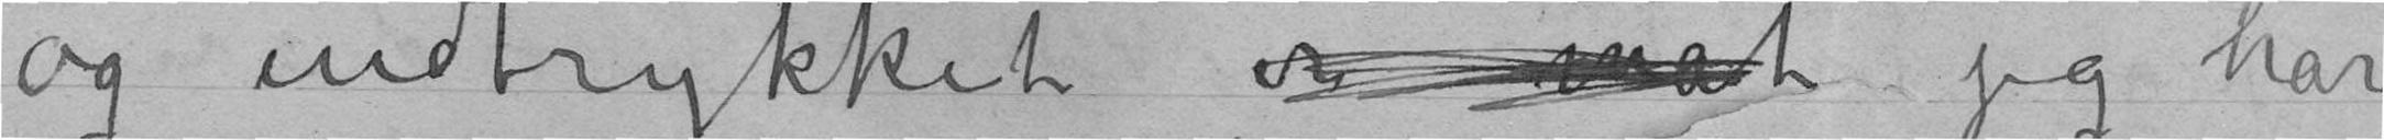og indtrykket er mat jeg har
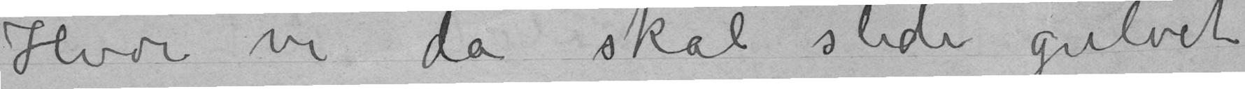Hvor vi da skal slide gulvet
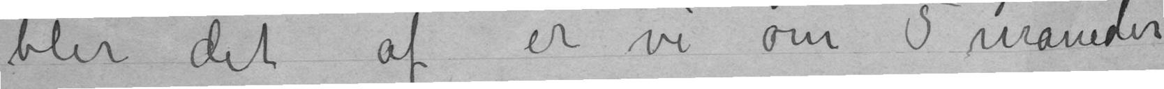blir det af er vi om 5 måneder
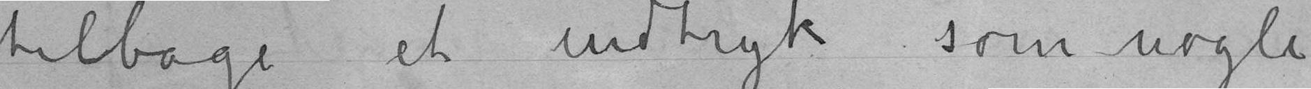tilbage et indtryk som nogle
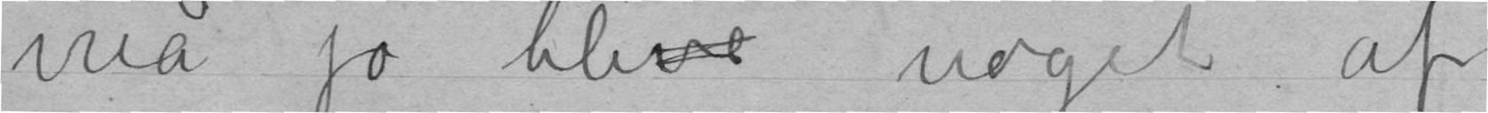må jo blive noget af
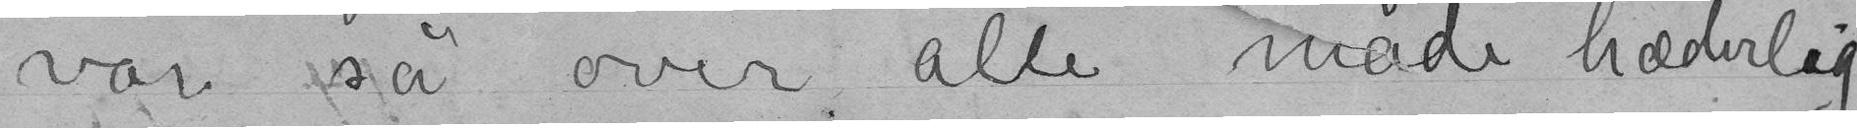var så over alle måde hæderlig
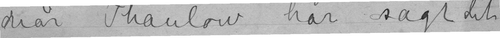når Thaulow har sagt det
